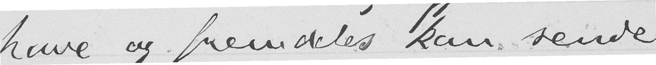have og fremdeles kan sende
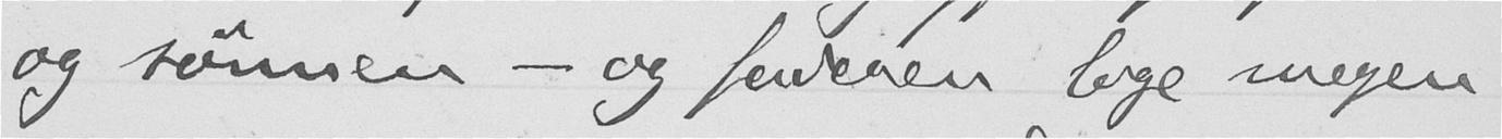og sønnen - og faderen lige megen
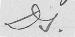Ds.
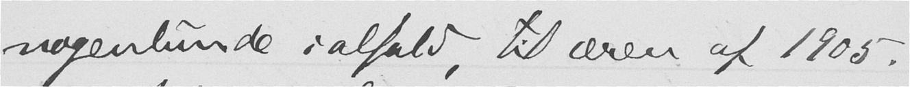nogenlunde ialfald, til æren af 1905.
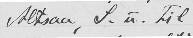Altsaa, S. u. til
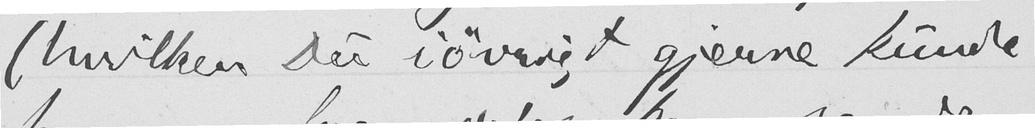(hvilken Du iøvrigt gjerne kunde
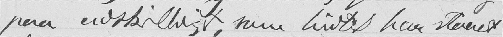paa adskilligt, som hidtil har staaet
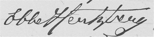Ebbe Hertzberg.
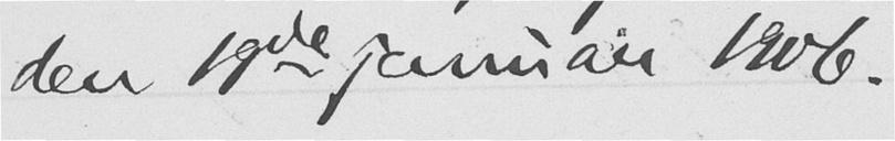den 19de januar 1906.
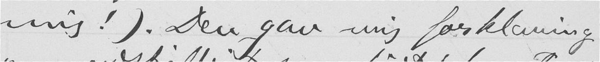mig!). Den gav mig forklaring
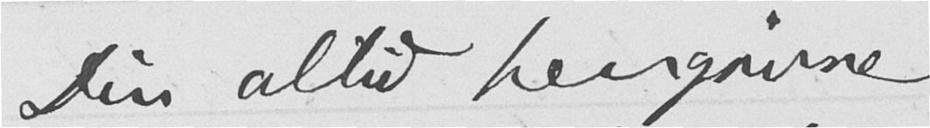Din altid hengivne
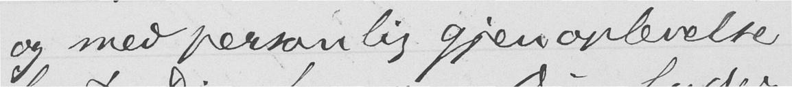og med personlig gjenoplevelse
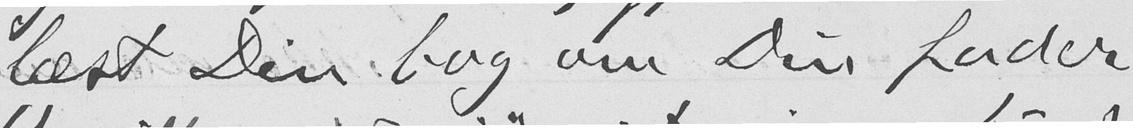læst Din bog om Din fader
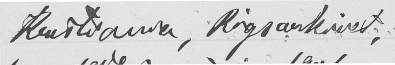Kristiania, Rigsarkivet,
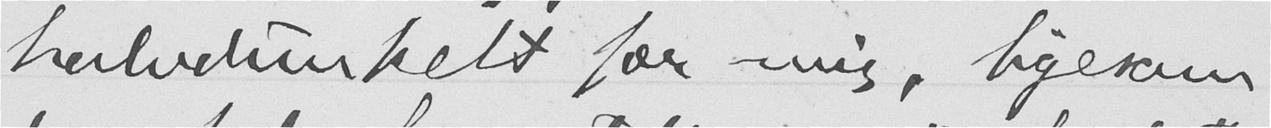halvdunkelt for mig, ligesom
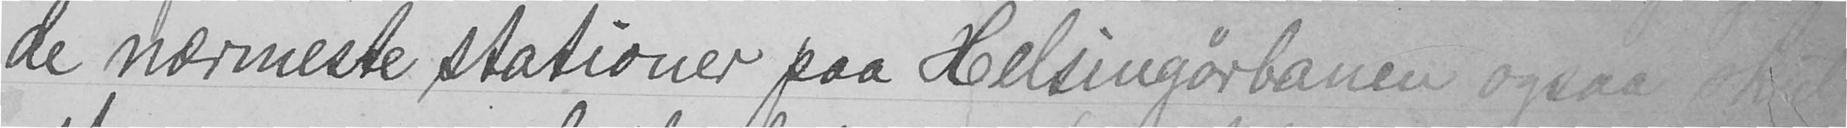de nærmeste stationer paa Helsingørbanen ogsaa skulde
